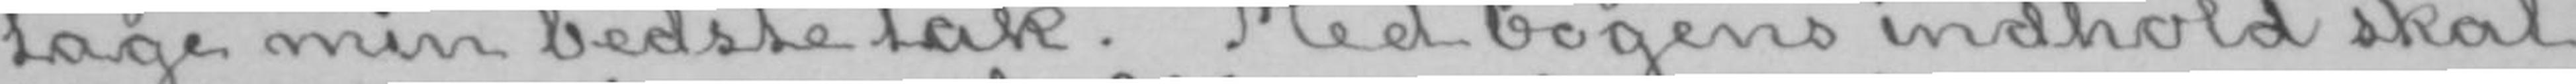tage min bedste tak. Med bogens indhold skal
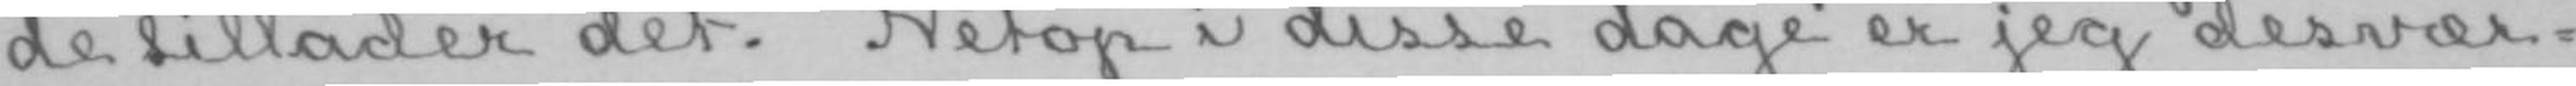de tillader det. Netop i disse dage er jeg desvær-
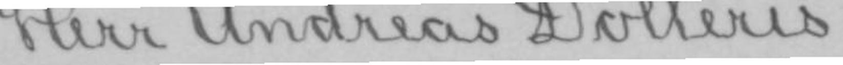Herr Andreas Dolleris.
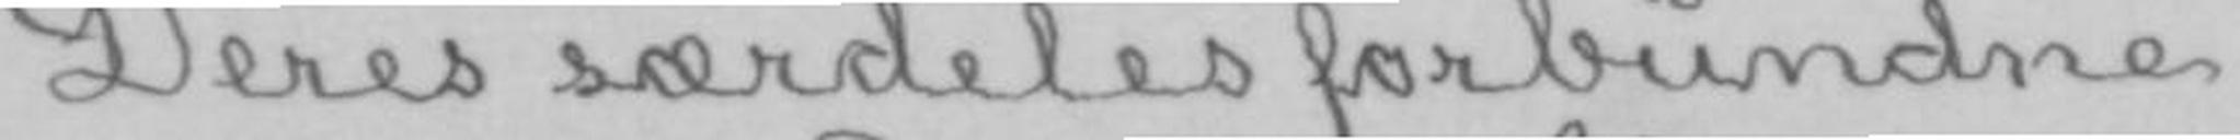Deres særdeles forbundne
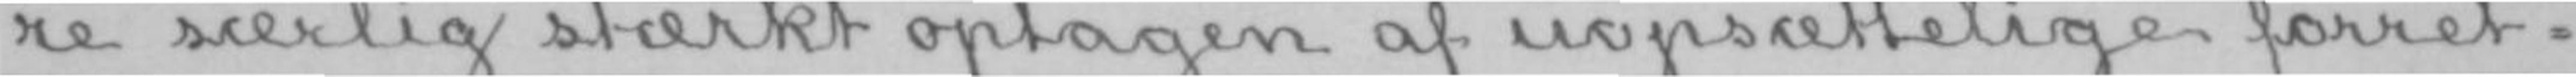re særlig stærkt optagen af uopsættelige forret-
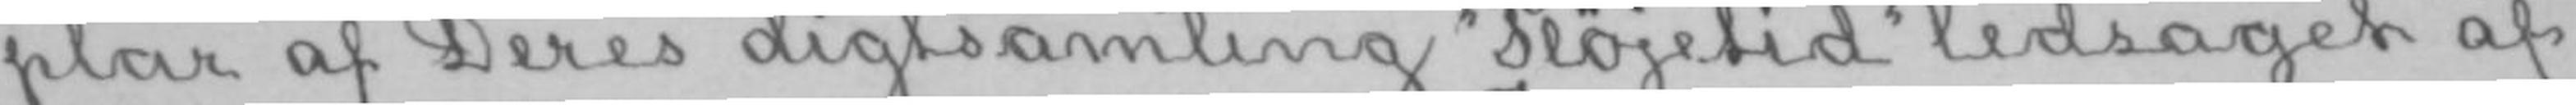plar af Deres digtsamling «Pløjetid» ledsaget af
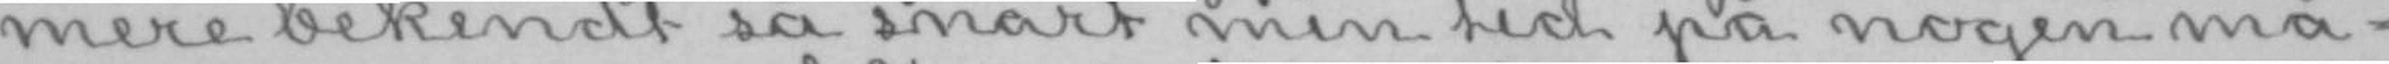mere bekendt så snart min tid på nogen må-
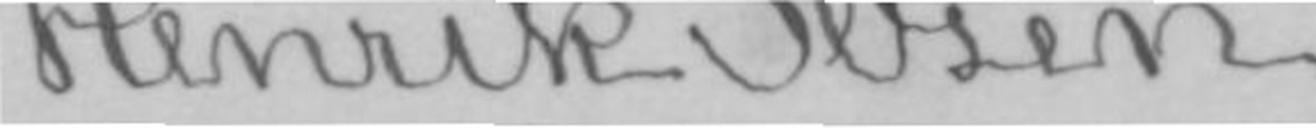Henrik Ibsen.
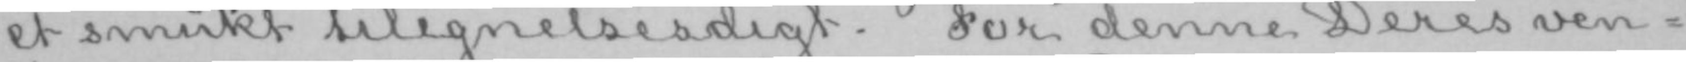et smukt tilegnelsesdigt. For denne Deres ven-
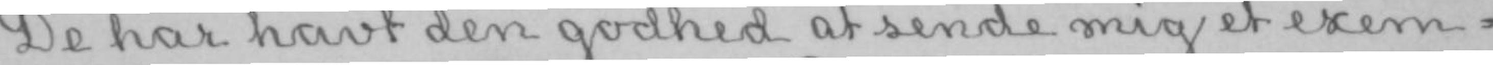De har havt den godhed at sende mig et exem-
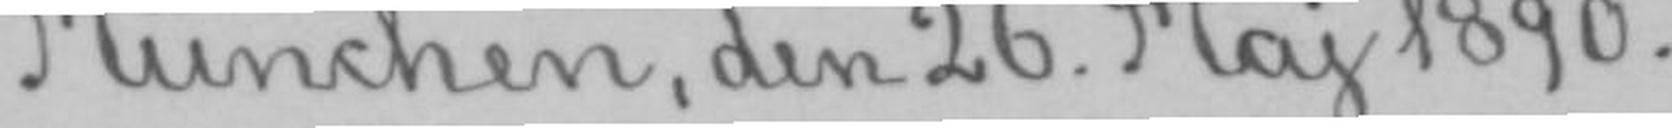München, den 26. Maj 1890.
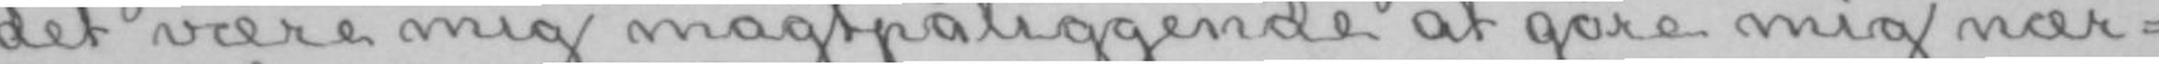det være mig magtpåliggende at gøre mig nær-
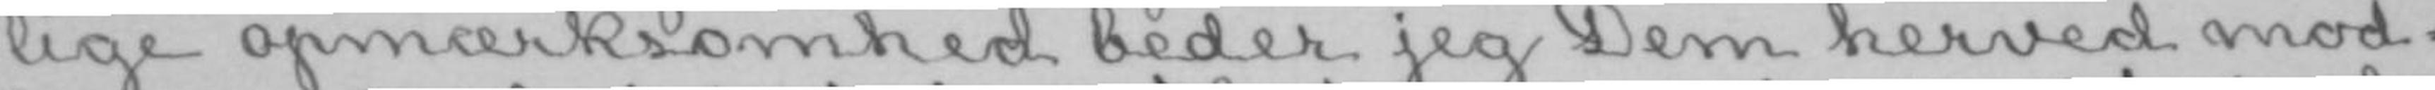lige opmærksomhed beder jeg Dem herved mod-
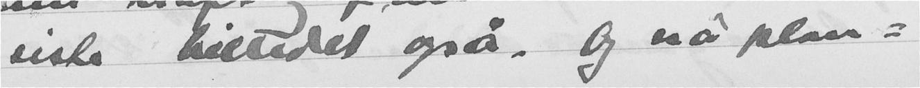siste billedet også. Og nå plan-
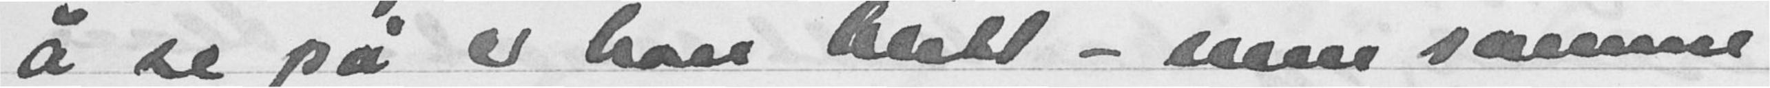å se på er han blitt - men samme
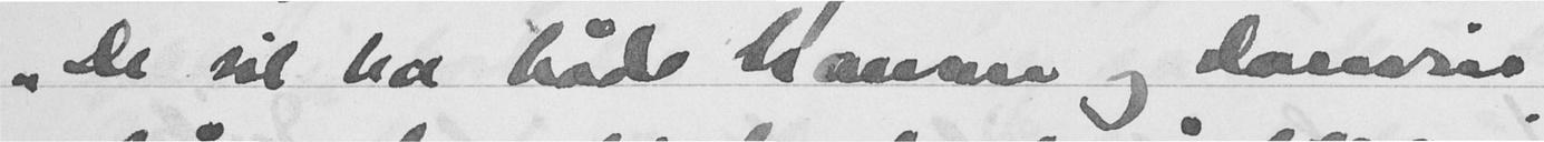"De vil ha både Nansen og darwin
